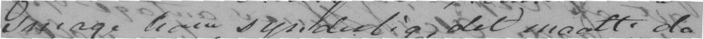smage ham synderlig, det maatte da
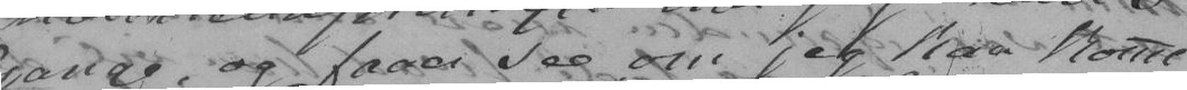Gange, og faaer see om jeg kan ko'me
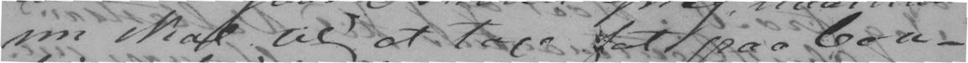nu skal til at tage fat paa Con-
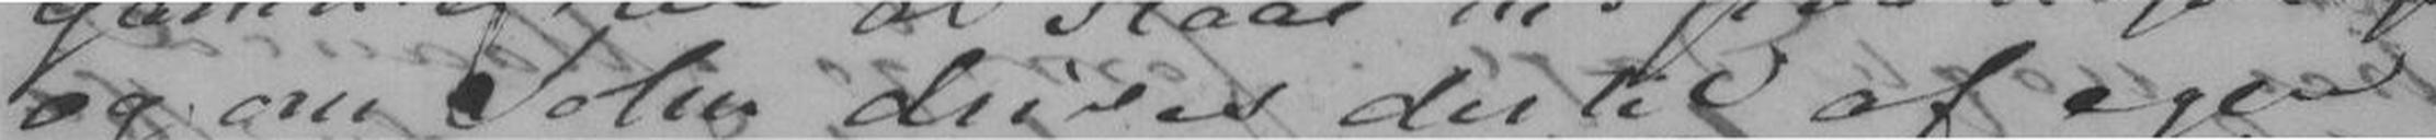og om John drives dertil af egen
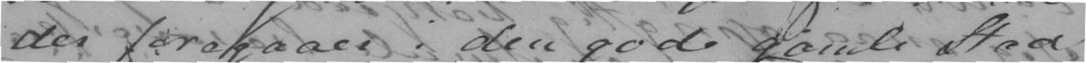der foregaaer i den gode gamle Stad
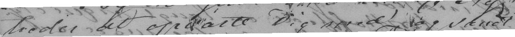heder at opvarte Dig med og sandt
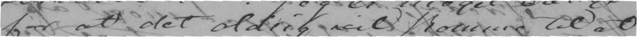for at det aldrig vil komme til at
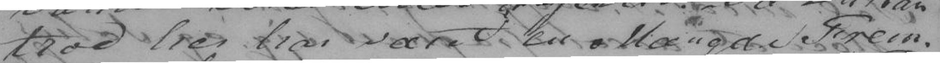troe her har været en Mængde Frem-
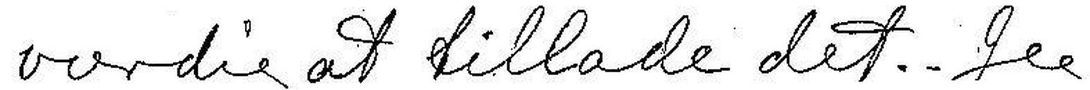værdig at tillade det. Jeg
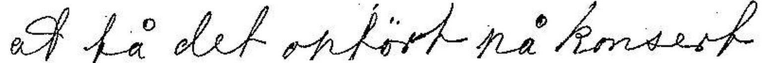at få det opført på konsert
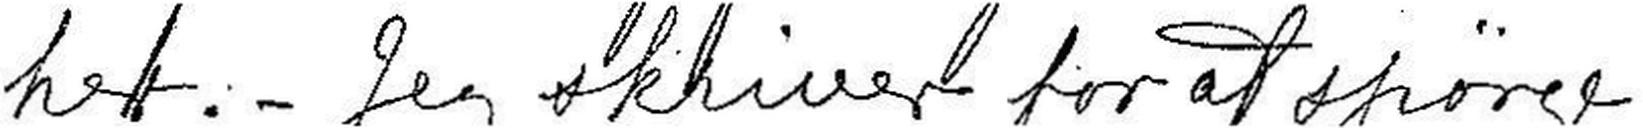her. - Jeg skriver for at spørge
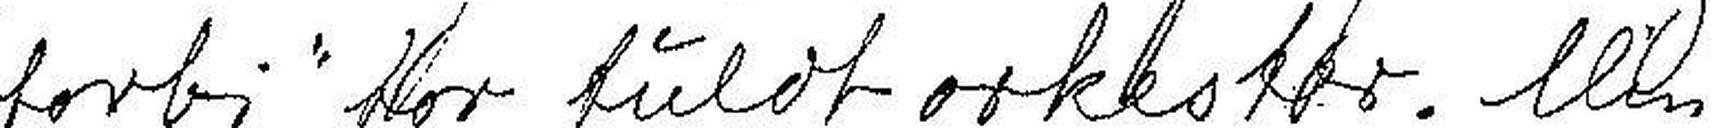forbi for fuldt orkester. Min
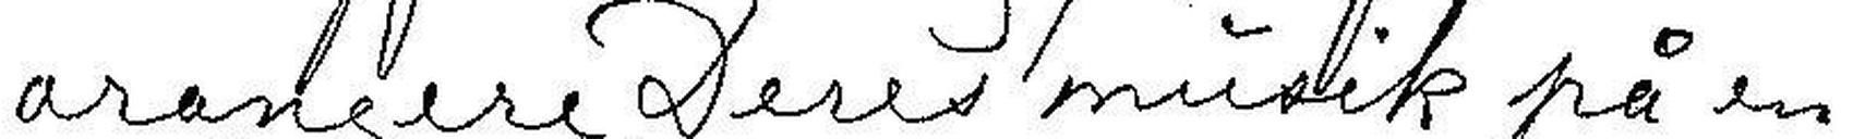arangere Deres musik på en
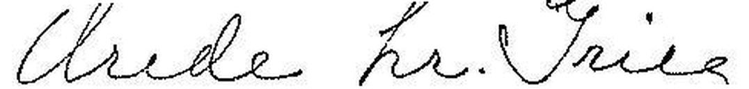Ærede hr. Greig!
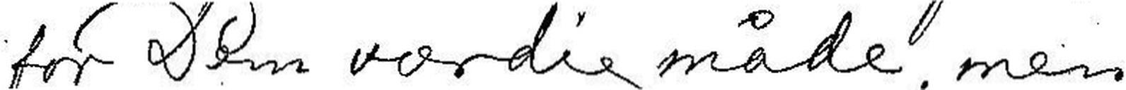for Dem værdig måde, men
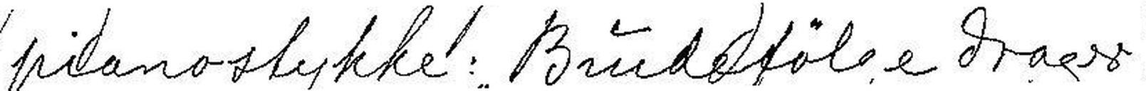Pianostykke: Brudefølge drager
